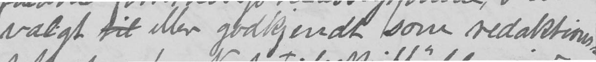valgt til eller godkjendt som redaktions-
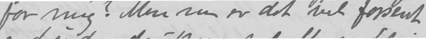for mig? Men nu er det vel forsent
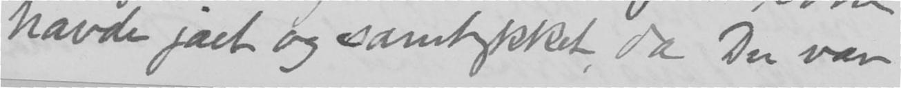havde jaet og samtykket, da Du var
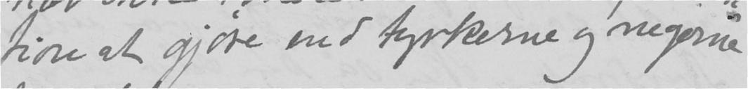tion at gjøre end tyrkerne og negerne
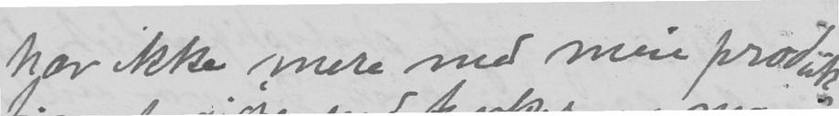har ikke mere med min produk-
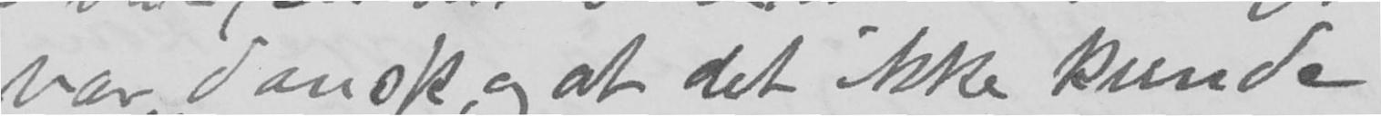var dansk, og at det ikke kunde
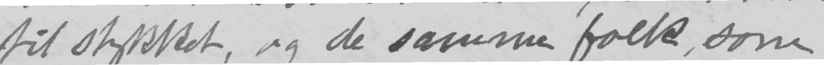til stykket, og de samme folk, som
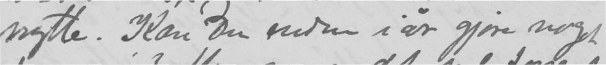nytte. Kan Du endnu i år gjøre noget
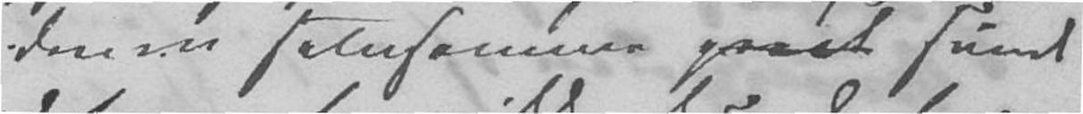denne selvsammes sunde
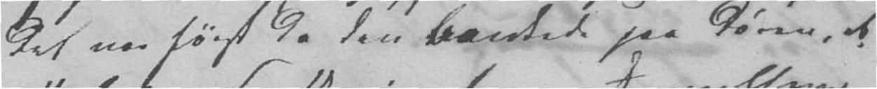Det var først da den bankede paa Døren, at
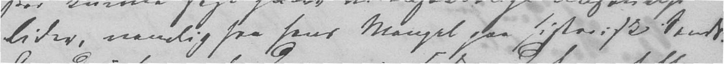lider, nemlig fra hans Mangel paa historisk Sands.
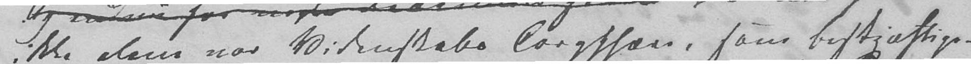ikke alene vor Videnskabs Coryphæer, som beskjæftige-
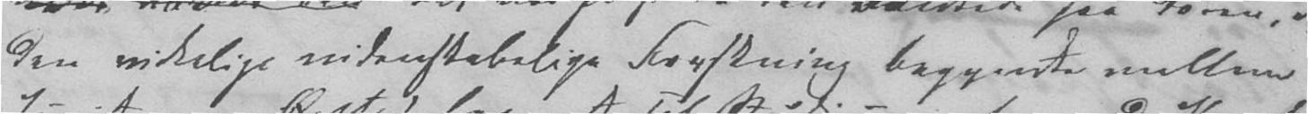den virkelige videnskabelige Forskning begyndte mellem
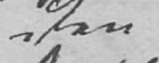Den
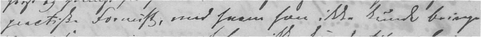practiske Fornuft, med hvem han ikke kunde bringe
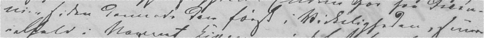nier siden dannede den først i Virkeligheden, senere
